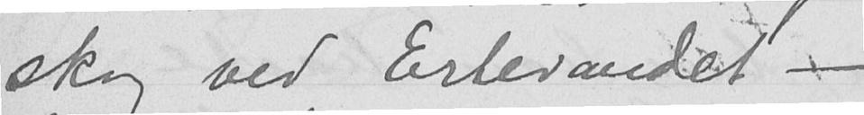skog ved Ertevandet -
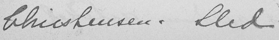Christensen. Sled
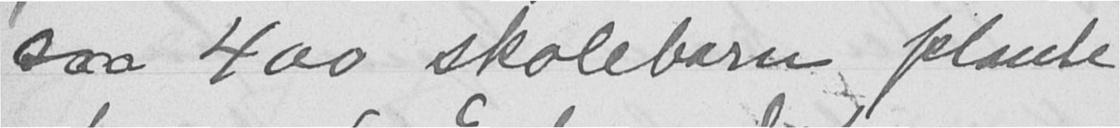saa 400 skolebarn plante
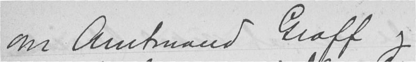om Amtmand Graff og
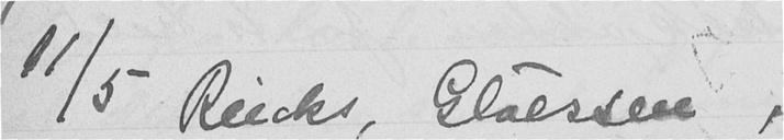11/5 Riecks, Gløersen,
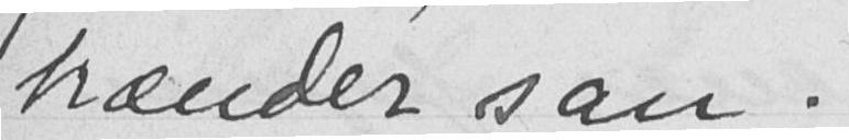brænder san.
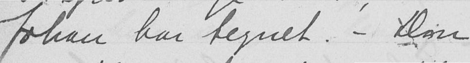Johan har tegnet - Don
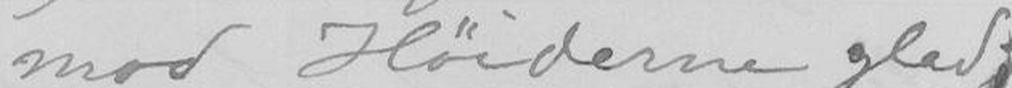mod Høiderne glad, -
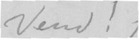(Vend!)
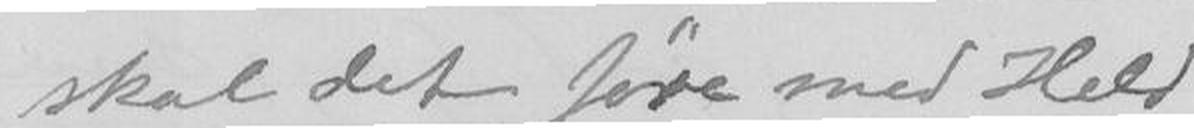skal det føre med Held
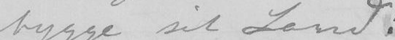bygge sit Land!
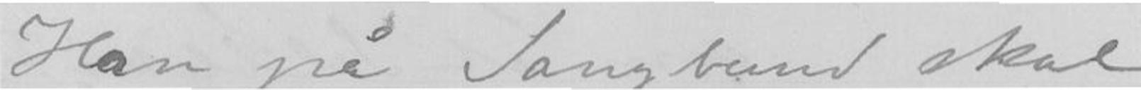Han på Sangbund skal
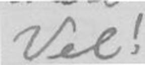Vel!
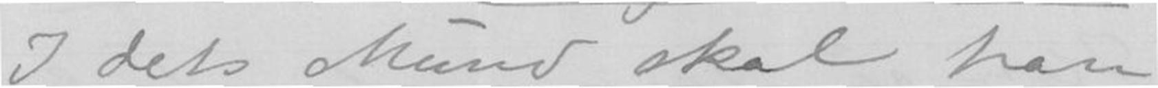I dets Mund skal Han
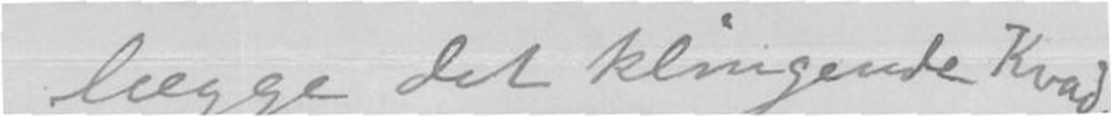lægge det klingende Kvad.
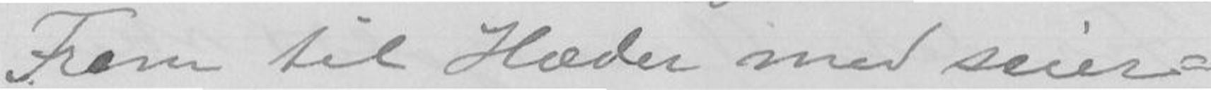Frem til Hæder med seier-
